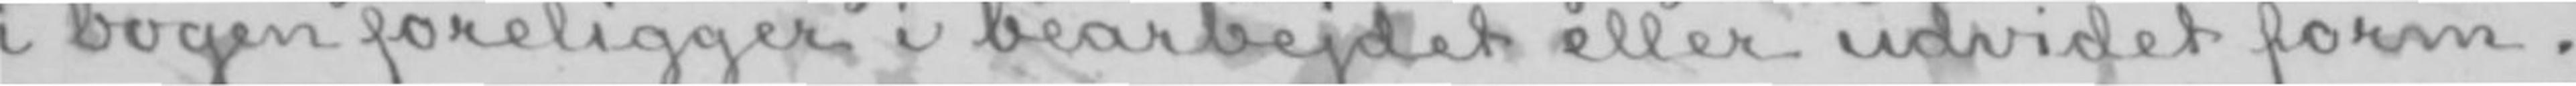i bogen foreligger i bearbejdet eller udvidet form.
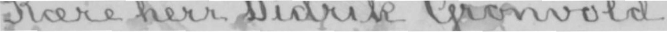Kære herr Didrik Grønvold,
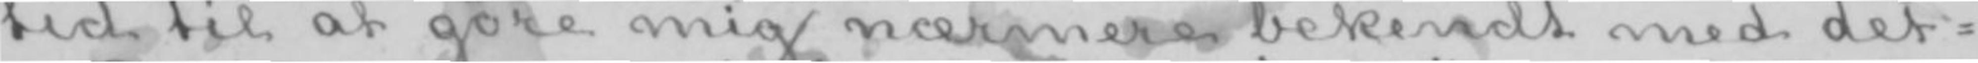tid til at gøre mig nærmere bekendt med det-
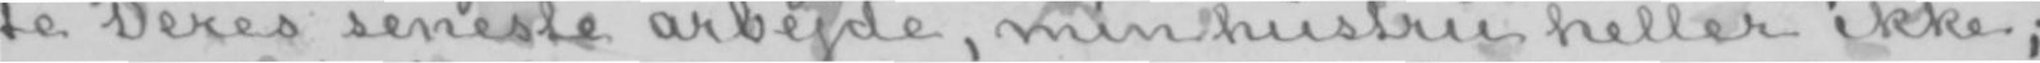te Deres seneste arbejde, min hustru heller ikke;
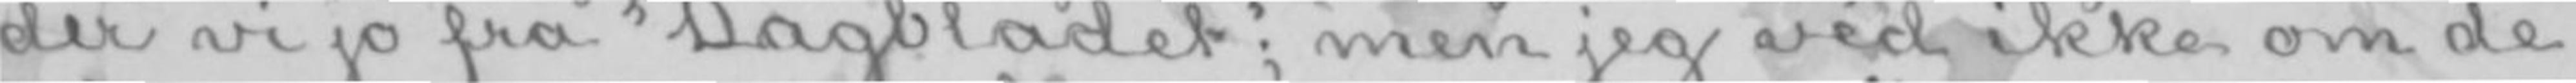der vi jo fra «Dagbladet»; men jeg véd ikke om de
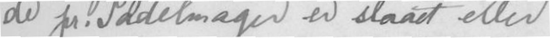de pr. Sadelmager er slaaet eller
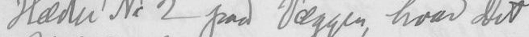Hæder No 2 paa Væggen, hvor Dit
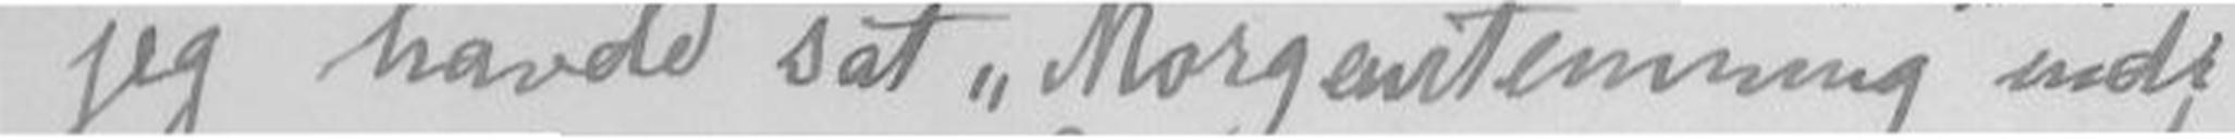jeg havde sat Morgenstemning inde,
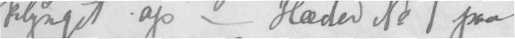klynget op - Hæder No 1 paa
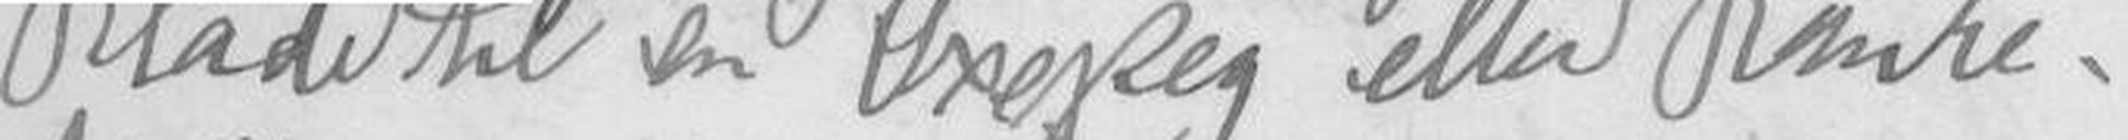Blade til en Oxesteg eller Banke-
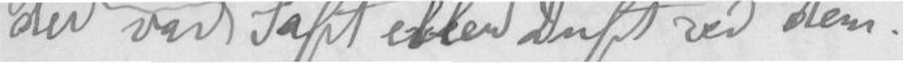der var hverken Saft eller Duft ved dem.
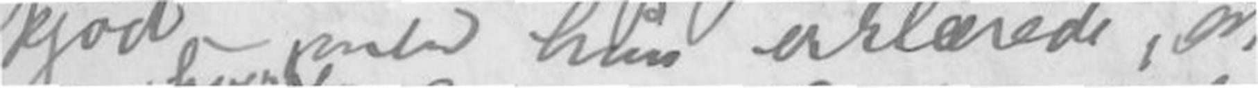kjød- men hun erklærede, at
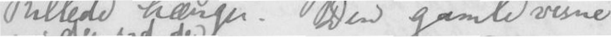Billede hænger. Den gamle visne
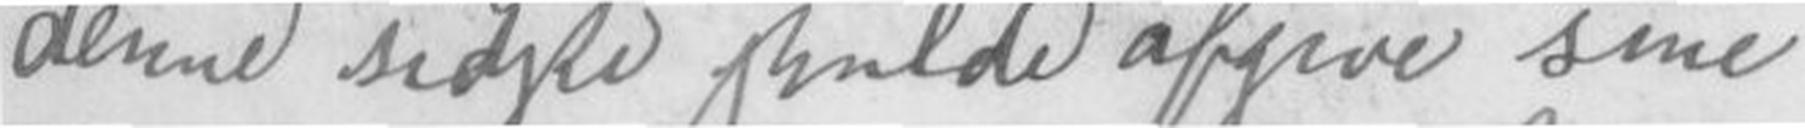denne sidste skulde af give sine
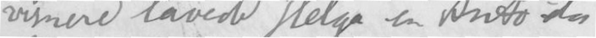visnere lovede Helga en Autoda-
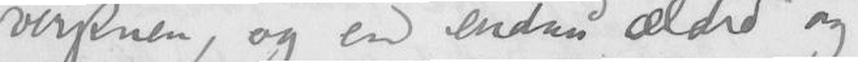verstuen, og en endnu ældre og
